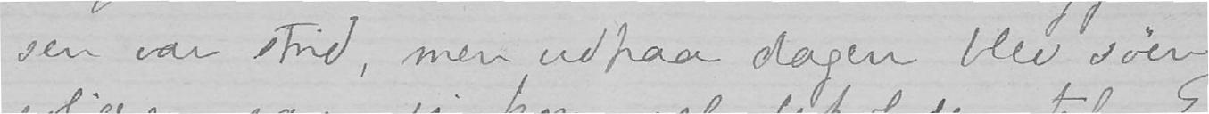sen var strid, men udpaa dagen blev søen
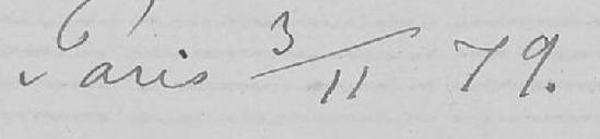Paris 3/11 79.
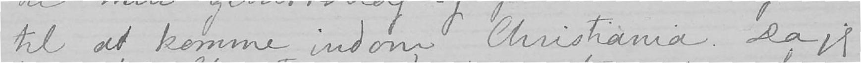til at komme indom Christiania. Da jeg
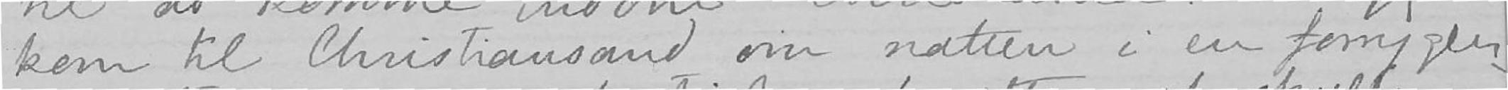kom til Christiansand om natten i en forrygen-
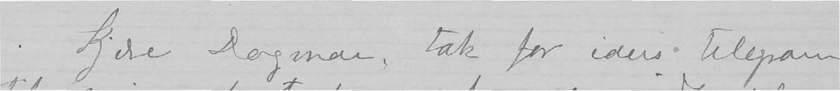Kjære Dagmar, tak for eders telegram
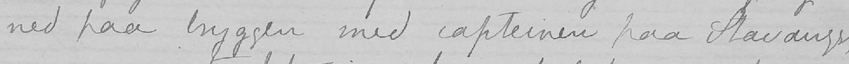ned paa bryggen med capteinen paa Stavanger,
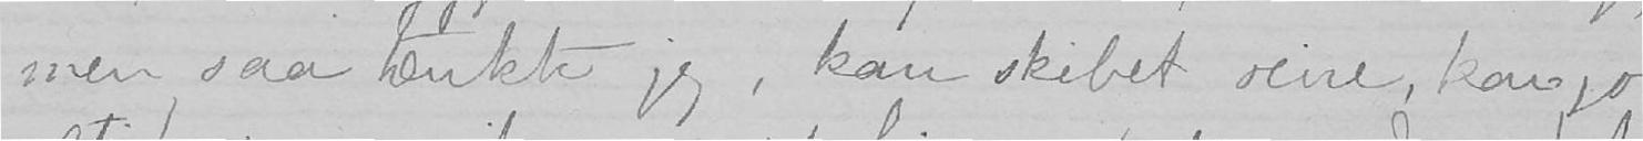men saa tænkte jeg, kan skibet reise, kan jo
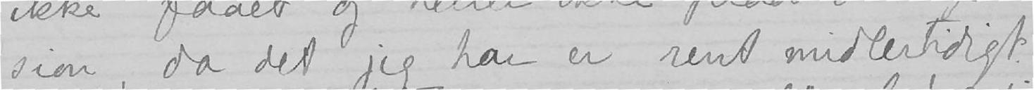sion, da det jeg har er rent midlertidigt.
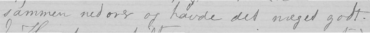sammen nedover og havde det meget godt.
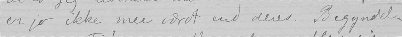er jo ikke mer værdt end deres. Begyndel-
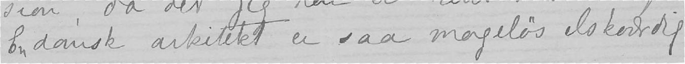En dansk arkitekt er saa mageløs elskværdig
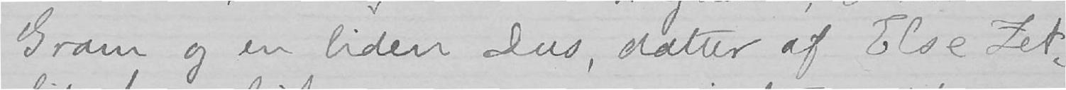Gram og en liden Dus, datter af Else Zet-
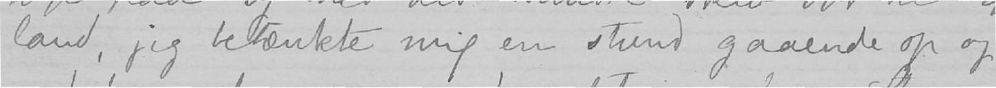land, jeg betenkte mig en stund gaaende op og
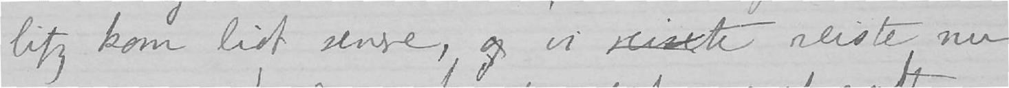litz kom lidt senere, og vi reisete reiste nu
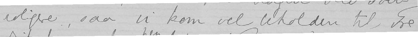roligere, saa vi kom vel beholden til Fre-
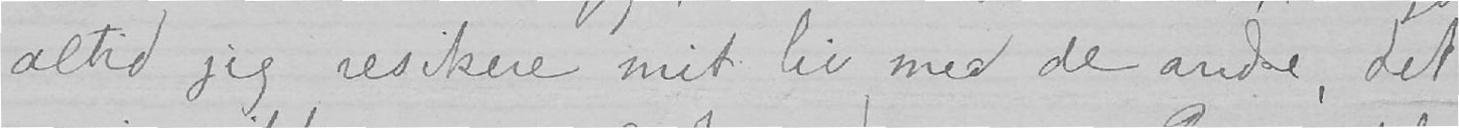altid jeg resikere mit liv med de andre, det
